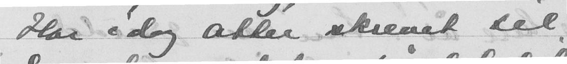Har idag atter skrevet til
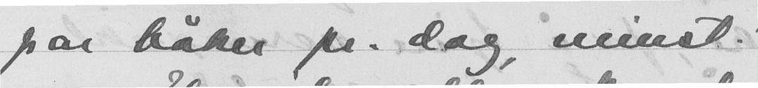par bøker pr. dag, minst!
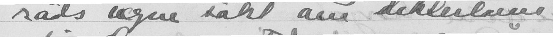råds vegne søkt om dikterlønn
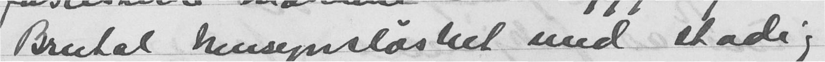Brutal hensynsløshet med stadig
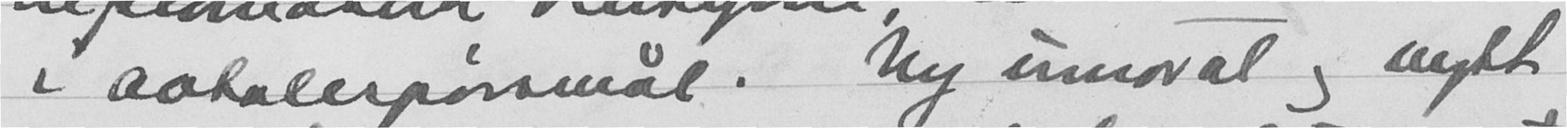i aatalerpørsmal. Ny umoral og nytt
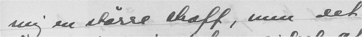mig en større straff, men det
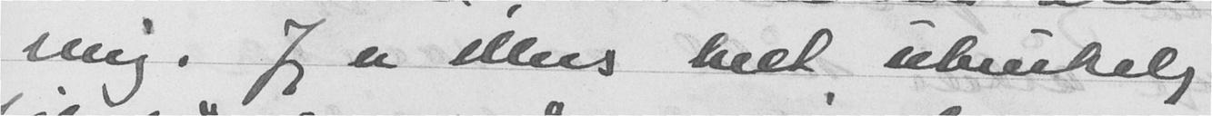mig. Jeg er ellers helt ubrukelig
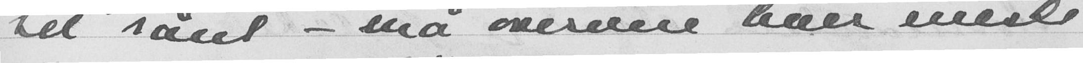til sånt - må overveie hver eneste
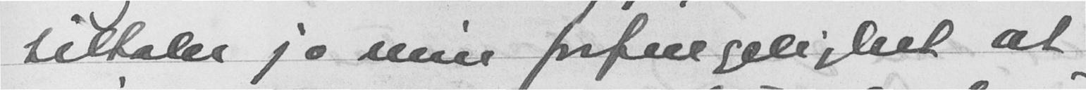tiltaler jo min forfengelighet at
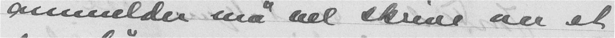ammelder må vel skrive om, et
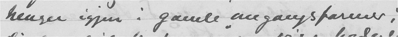henger igjen i gamle "omgangsformer,"
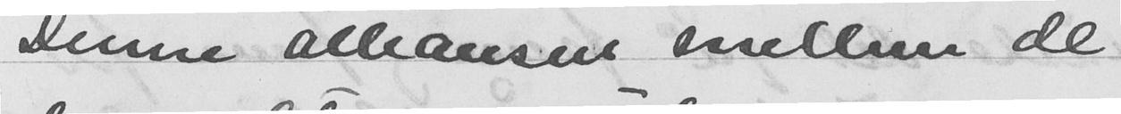Denne alliansen mellem de
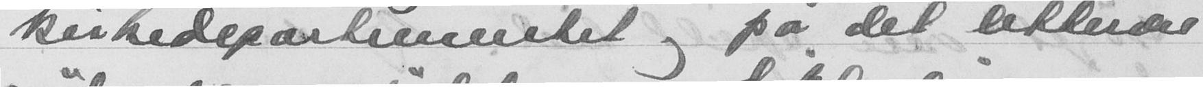kirkedepartementet og på det litterære
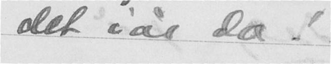det iår da!
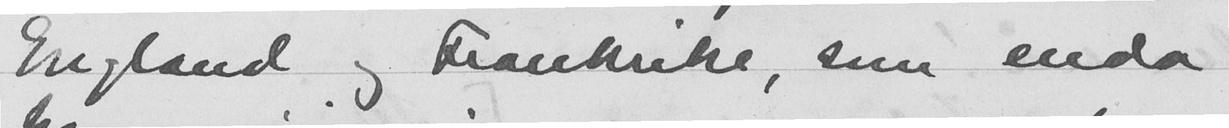England og Frankrike, som enda
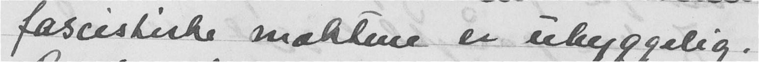fascistiske maktene er uhyggelig.
